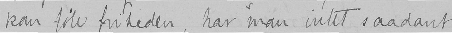kan føle friheden, har man intet saadant
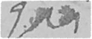gaa
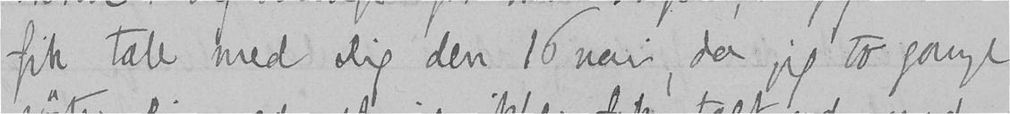fik tale med Dig den 16 mai, da jeg to gange
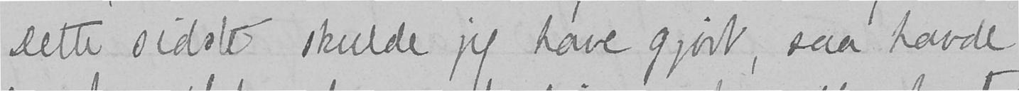Dette sidste skulde jeg have gjort, saa havde
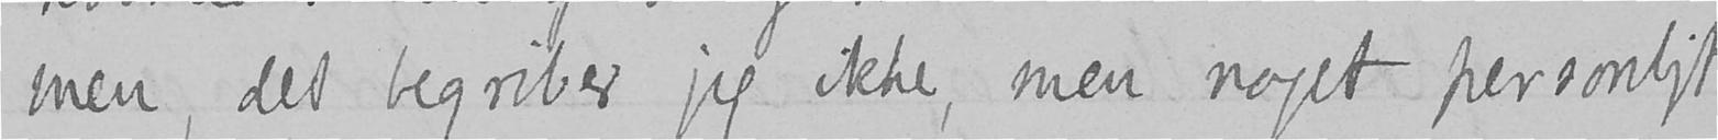men, det begriber jeg ikke, men noget personligt
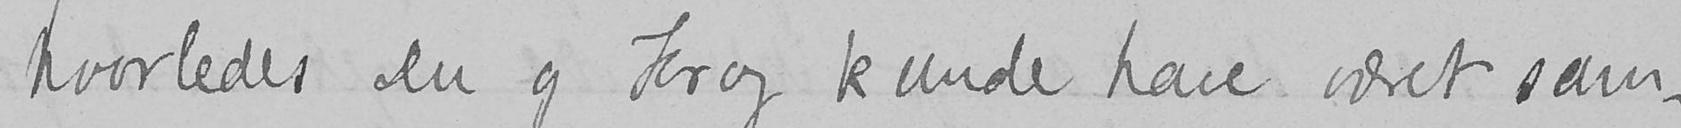hvorledes Du og Krog kunde have været sam-
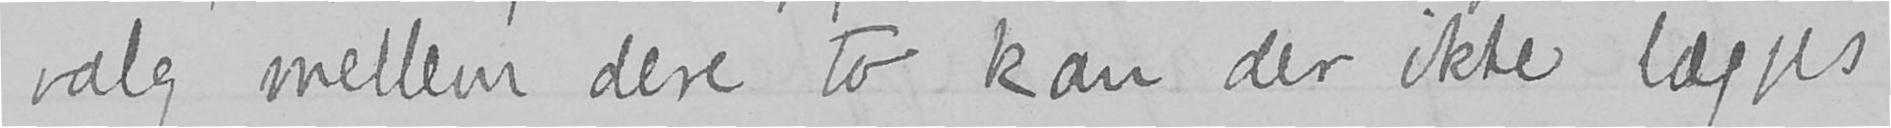valg mellem dere to kan der ikke lægges
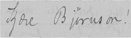Kjære Bjørnson!
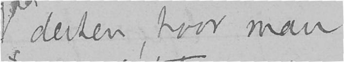derhen, hvor man
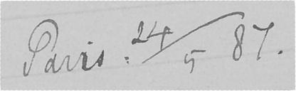Paris 24/5 87.
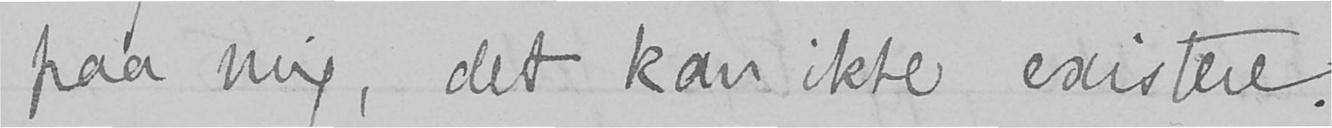paa mig, det kan ikke existere.
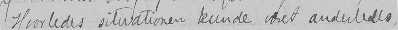Hvorledes situationen kunde været anderledes,
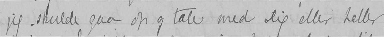jeg skulde gaa op og tale med Dig eller heller
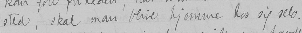sted, skal man blive hjemme hos sig selv.
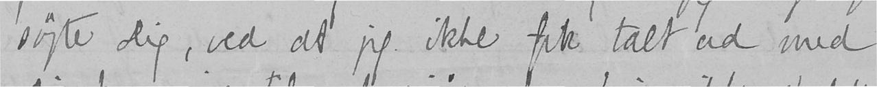søgte Dig, ved at jeg ikke fik talt ud med
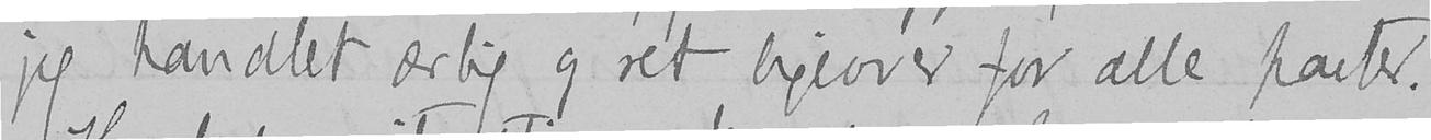jeg handlet ærlig og ret ligeover for alle parter.
